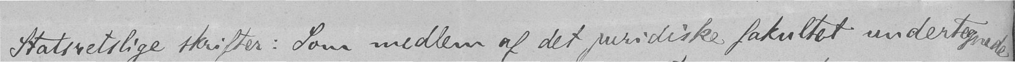Statsretslige skrifter: Som medlem af det juridiske fakultet undertegnet
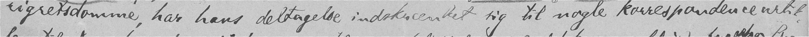rigretsdomme, har hans deltagelse indskrænket sig til nogle korrespondenceartik-
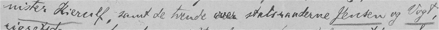nister Kierulf, samt de tvende over statsraaderne Jensen og Vogt,
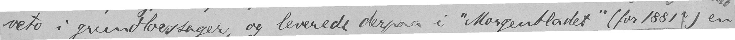veto i grundlovssager, og leverede derpaa i "Morgenbladet" (for 1881?) en
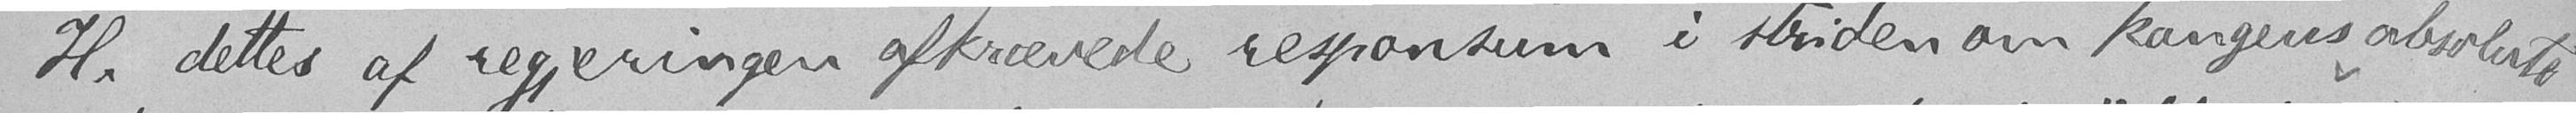H. dettes af regjeringen afkrævede responsum i striden om kongens absolute
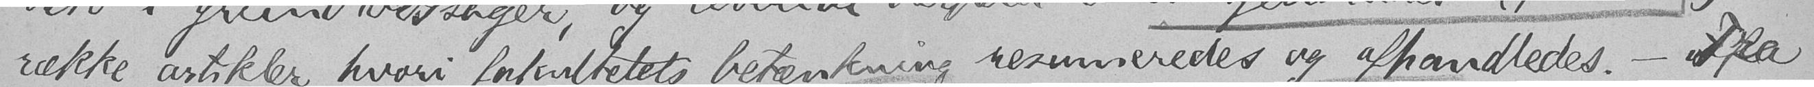række artikler, hvori fakultetets betænkning resumeredes og afhandledes. - Fra
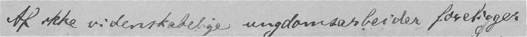Af ikke videnskabelige ungdomsarbeider foreligger
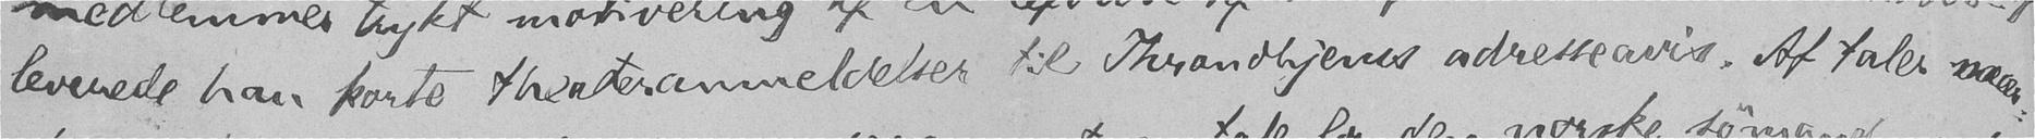leverede han korte theateranmeldelser til Throndhjems adresseavis. Af taler mær-
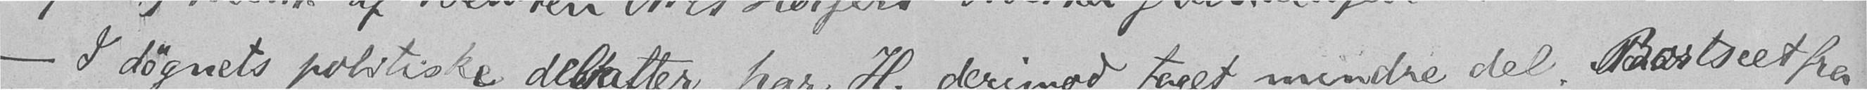- I døgnets politiske debatter har H. derimod taget mindre del. Bortseet fra
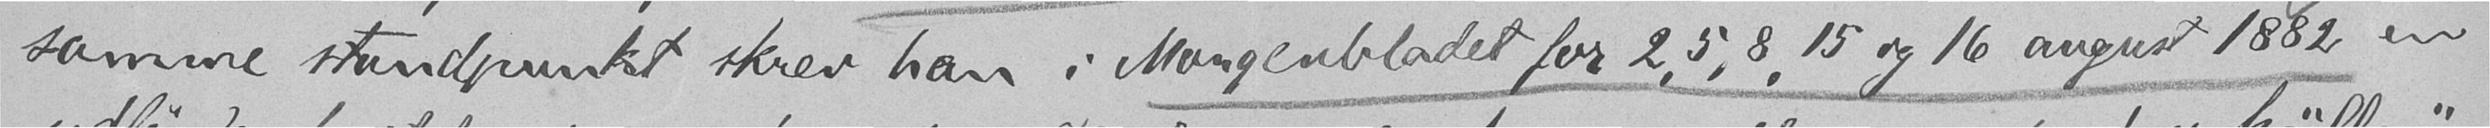samme standpunkt skrev han i Morgenbladet for 2, 5, 8, 15 og 16 august 1882 en
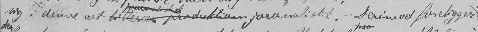sig i denne art  journalistik. - Derimod foreligger
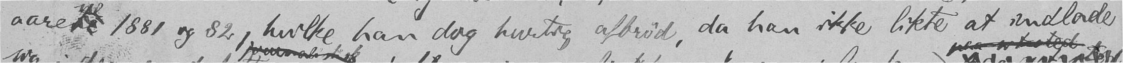aarene 1881 og 82, hvilke han dog hurtig afbrød, da han ikke likte at indlade
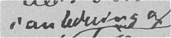i anledning af
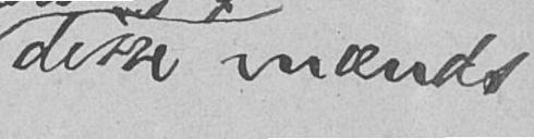disse mænds
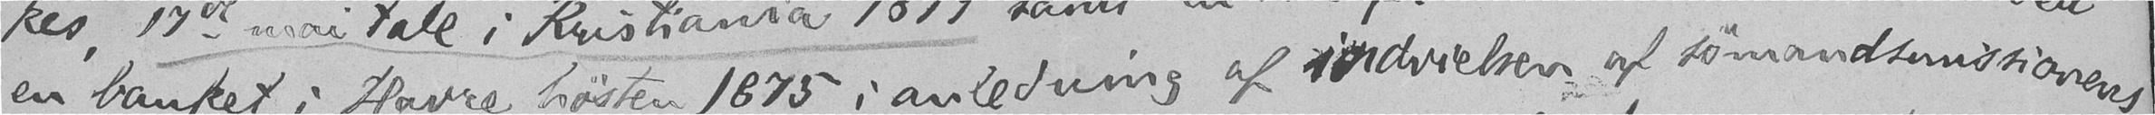en banket i Havre høsten 1875 i anledning af indvielsen af sømandsmissionens
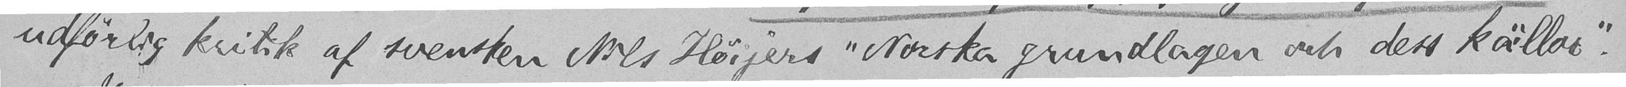udførlig kritik af svensken Nils Höijers "Norska grundlagen och dess källor".
